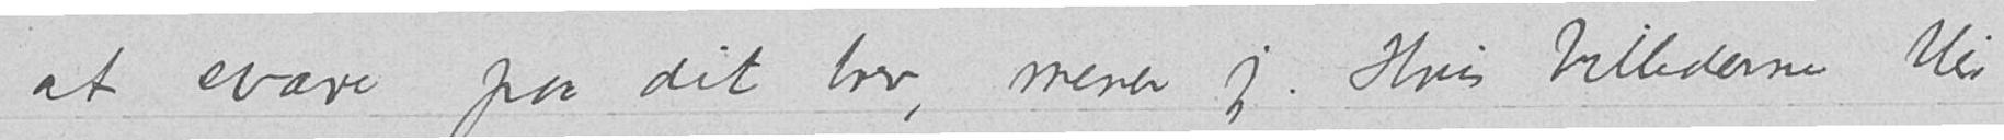at svare paa dit brev, mener jeg. Hvis billederne blir
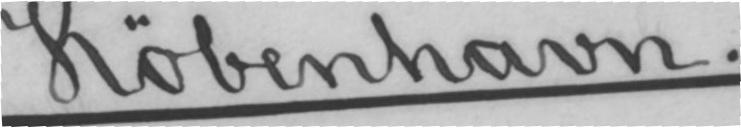København.
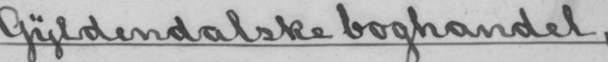Gyldendalske boghandel,
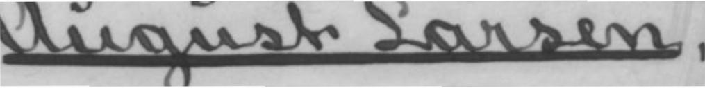August Larsen,
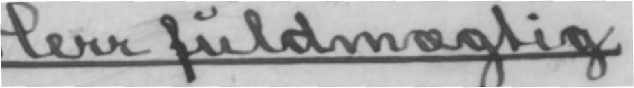Herr fuldmægtig
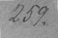259.
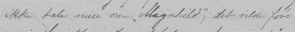ikke tale mere om "Magnhild"; det vilde føre
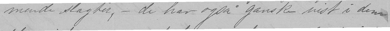mende slægter, - de har også ganske vist i dem
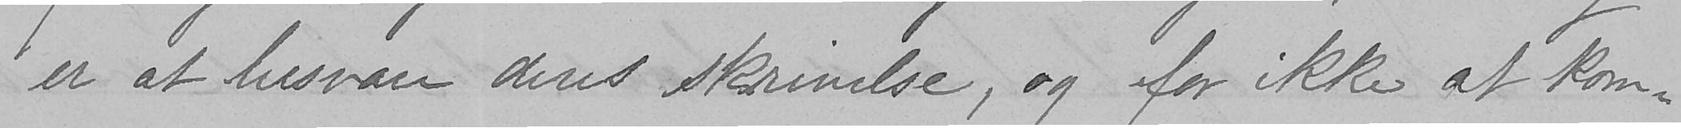er at besvare deres skrivelse, og for ikke at kom-
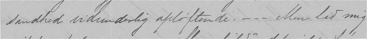sandhed vidunderlig opløftende. - - - Men lad mig
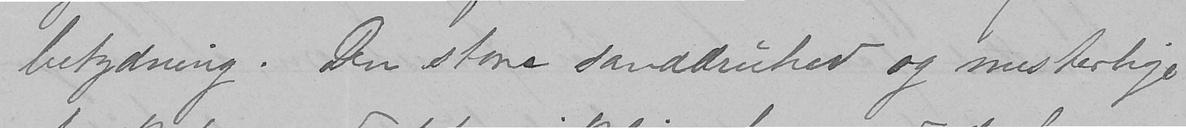betydning. Den store sanddruhed og mesterlige
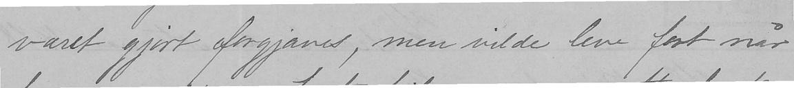været gjort forgjæves, men vilde leve først når
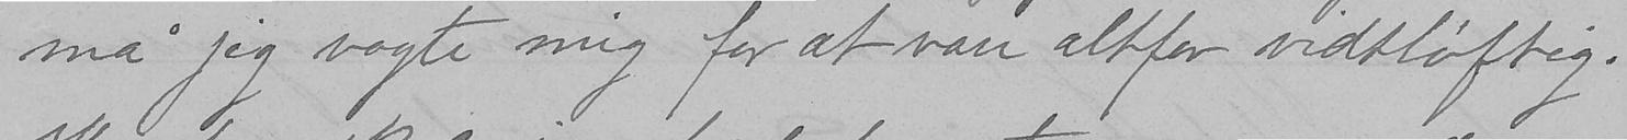må jeg vogte mig for at være altfor vidtløftig.
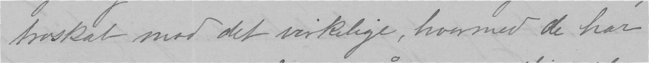troskab mod det virkelige, hvormed de har
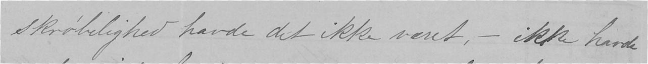skrøbelighed havde det ikke været, - ikke havde
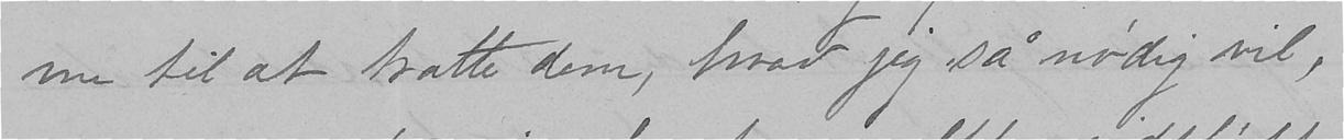me til at trætte dem, hvad jeg så nødig vil,
